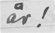år!
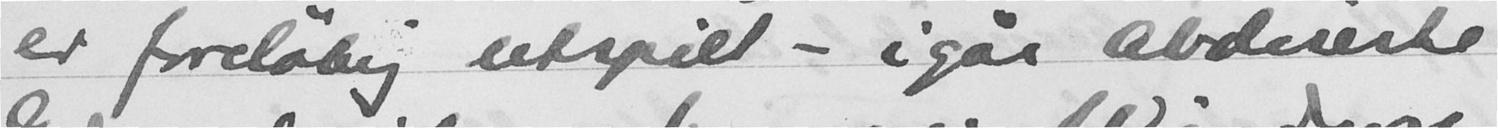er foreløbig utspilt - igår abdiserte
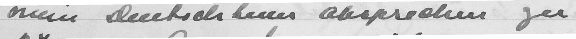men Deietschhuns atsprechen gir
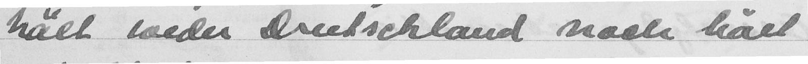huet seder Dretschland naate hait
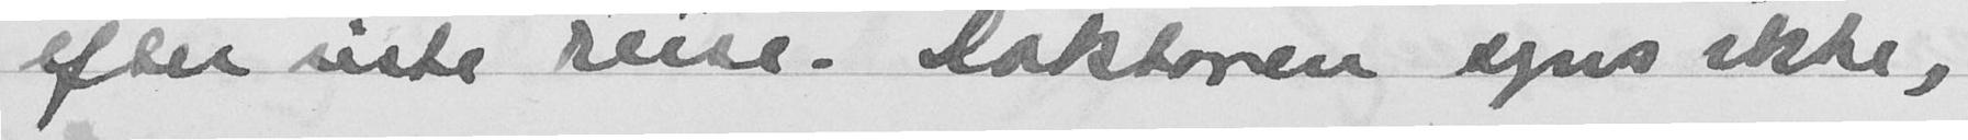efter siste reise. Doktoren syns ikke,
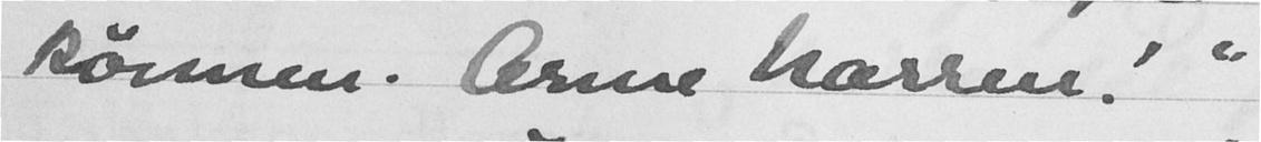kømm. Arme karren!
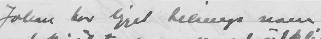Johan har ligget tilsengs noen
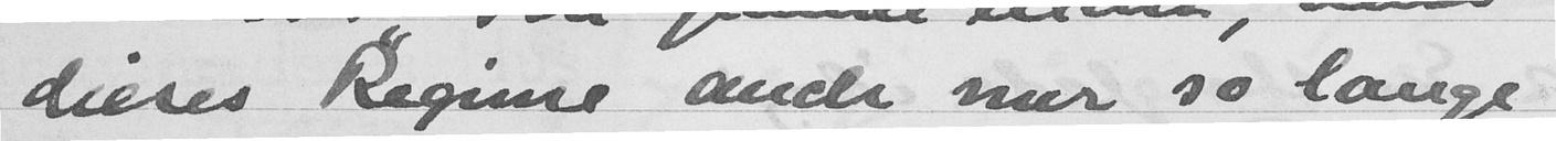dieses Regime anchr mer so lange
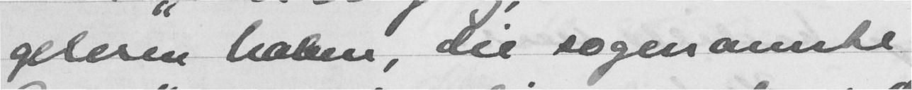gelesen haben, Du sogenanisté
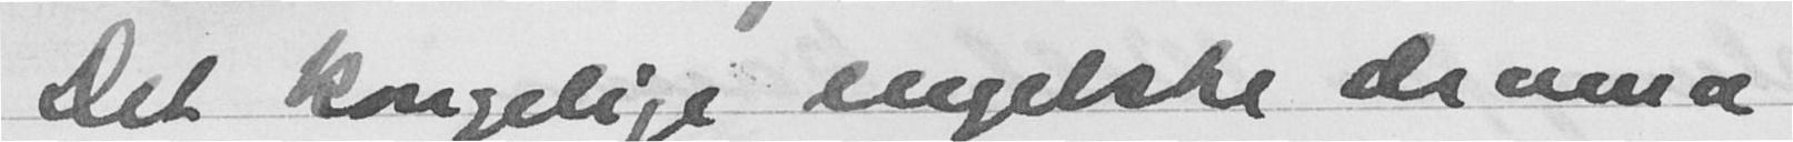Det kongelige engelske drama
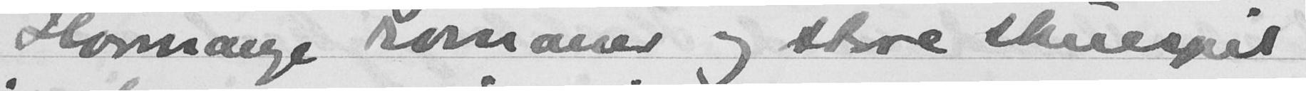Hvormange romaner og store skuespil
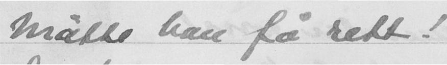Måtte han få rett!
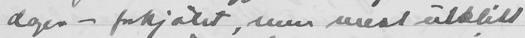dager - forkjølet, men mest utslitt
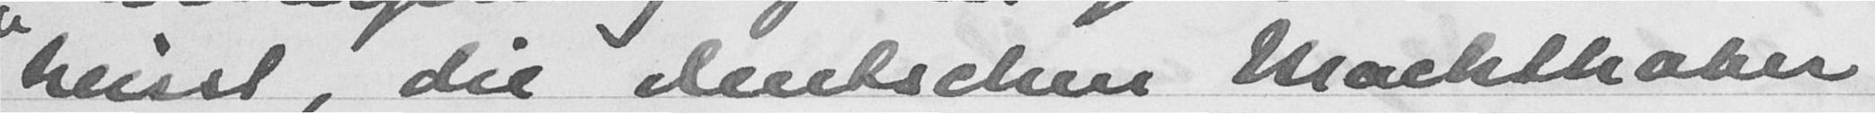henst, die deutschen Mockthaber
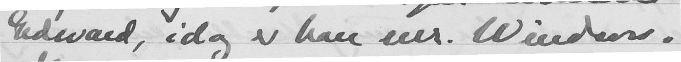Edvard, i dag er han mr. Windsor.
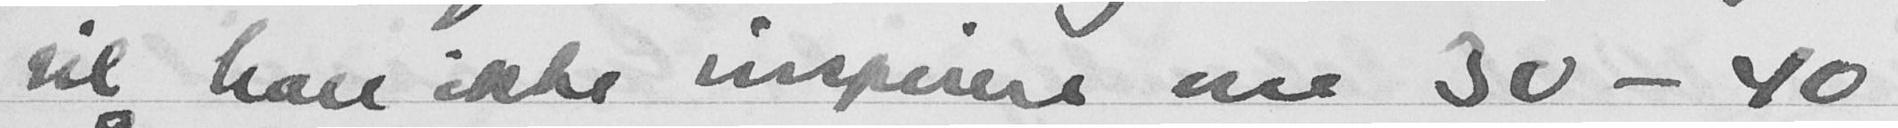vil han ikke inspirere om 30 - 40
